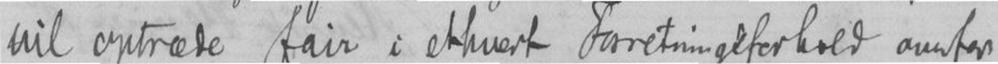vil optræde fair i ethvert forretningsforhold overfor
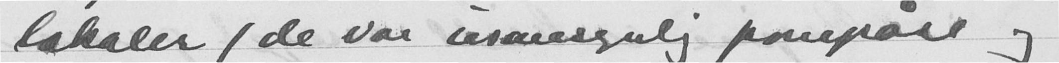lokaler (de var usansynlig pompøse og
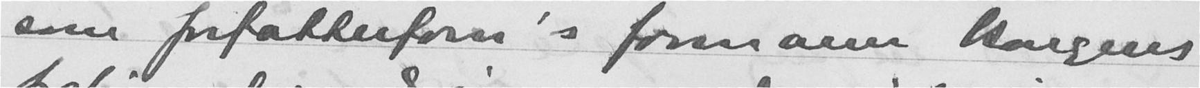som forfattesforn's formann kongens
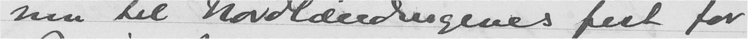in til Nordlændingenes fest for
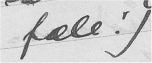fæle!)
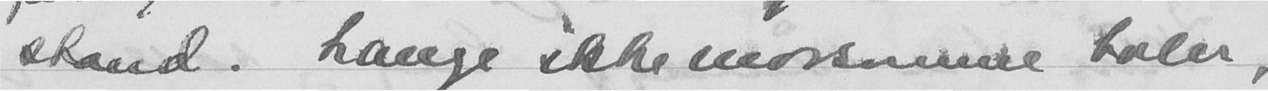stand. Lange ikke morsomme taler,
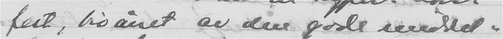fest, bivånet av den gode middel-
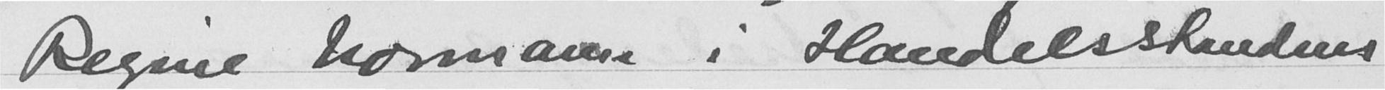Regine Normann i Handelsstandens
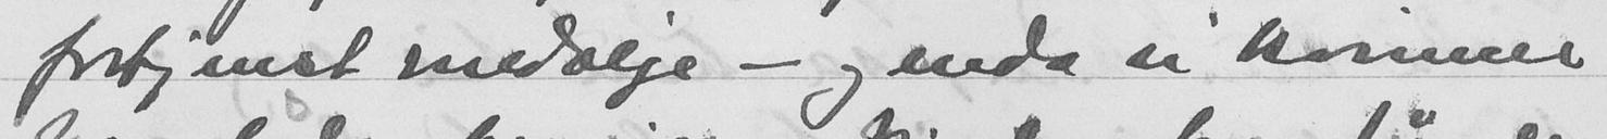fortjenst medalje - og enda vi kvinner
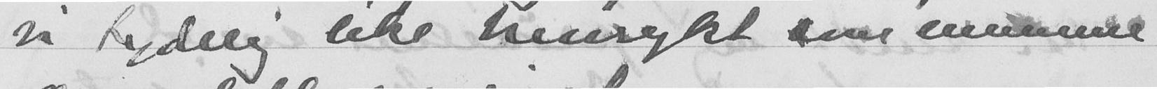vi tydelig like henrykt som mennene,
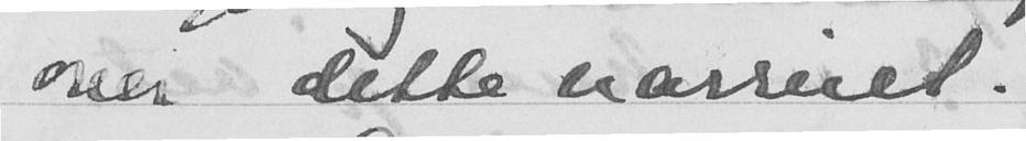over dette narreiet!
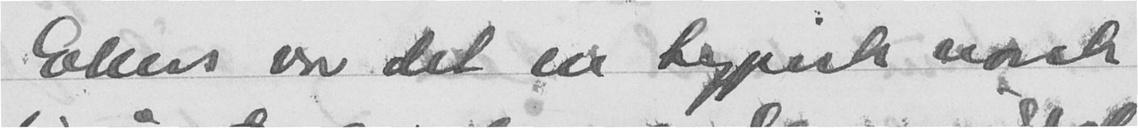Ellers var det en typisk norsk
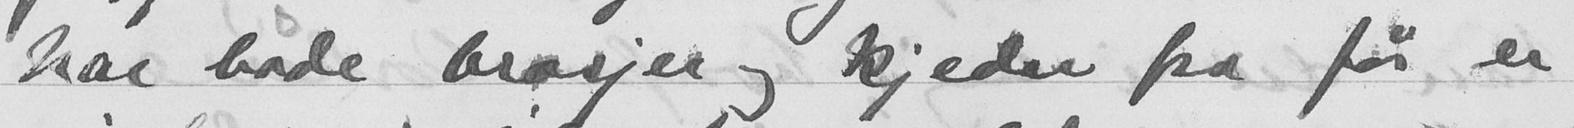har både brasjer og kjeder fra før er
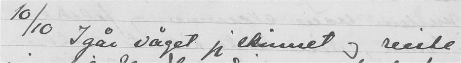10/10 Igår våget jeg skinnet og reiste
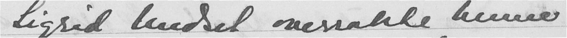Sigrid Undset overrakte henne
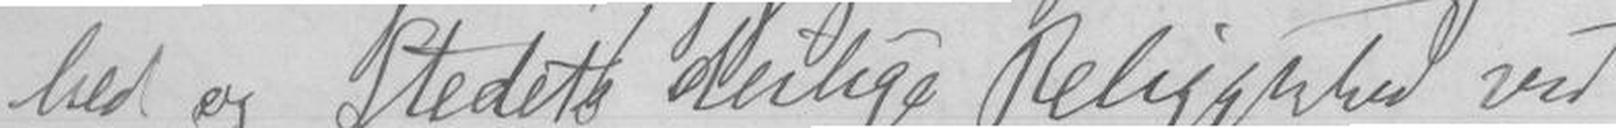hed og Stedets deilige Beliggenhed ved
